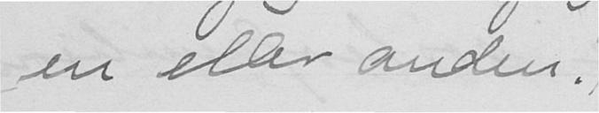en eller anden.)
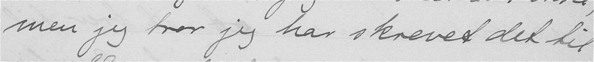men jeg tror jeg har skrevet det til
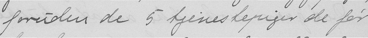foruden de 5 tjenestepiger de før
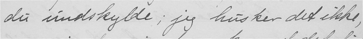du undskylde; jeg husker det ikke
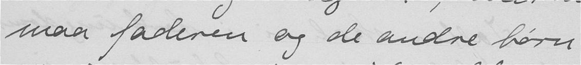maa faderen og de andre børn
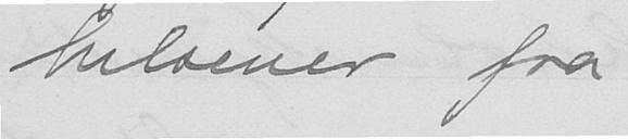hilsener fra
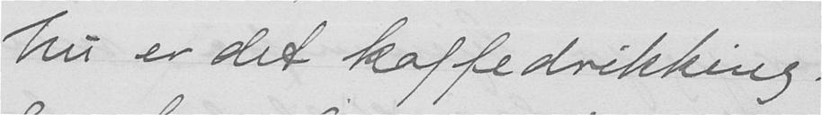Nu er det kaffedrikking.
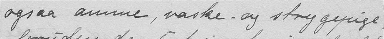ogsaa amme, vaske- og strygepige
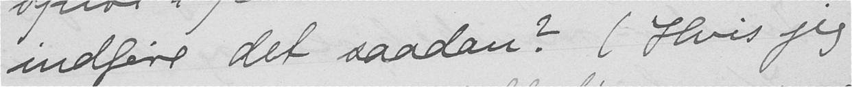indføre det saadan? (Hvis jeg
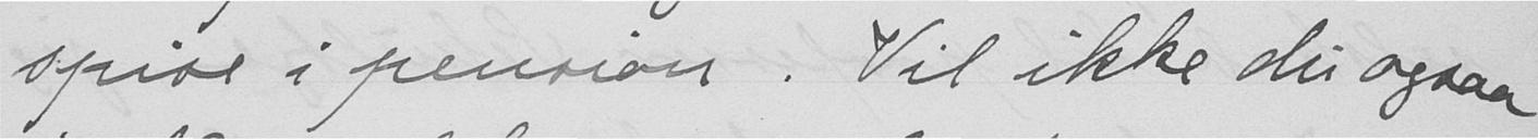spise i pension. Vil ikke du ogsaa
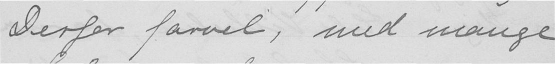Derfor farvel, med mange
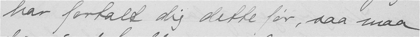har fortalt dig dette før, saa maa
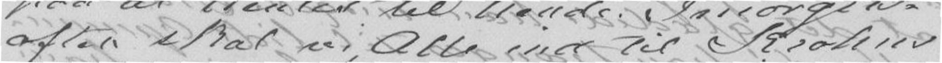aften skal vi Alle ind til Krohns
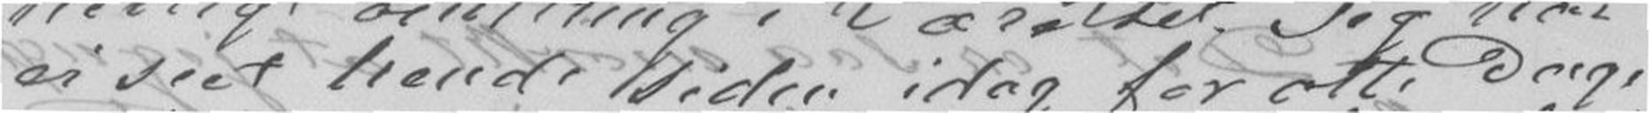ei seet hende siden idag for otte Dage
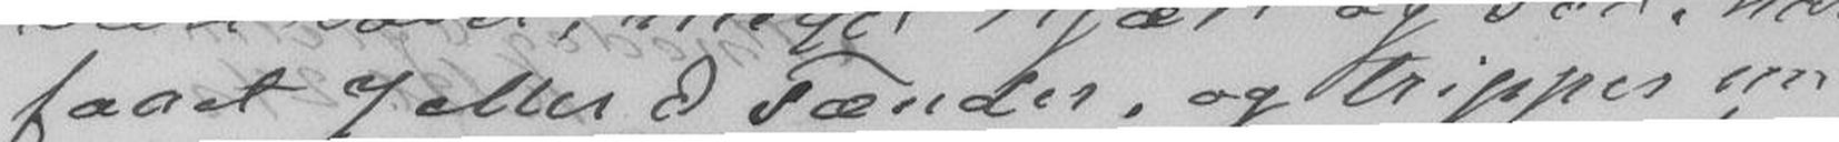faaet 7 eller 8 Tænder, og tripper nu
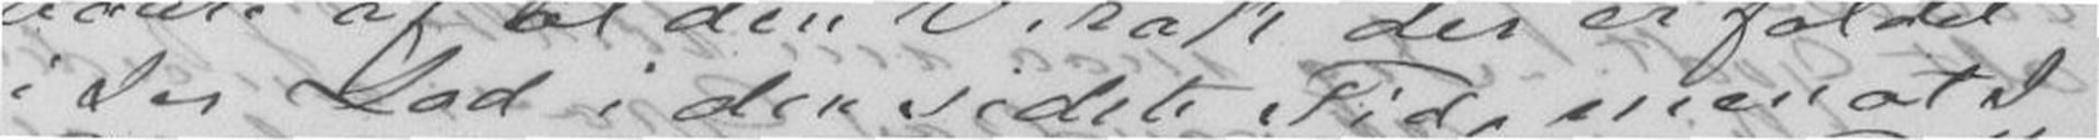i Jer Lod i den sidste Tid, men at I
